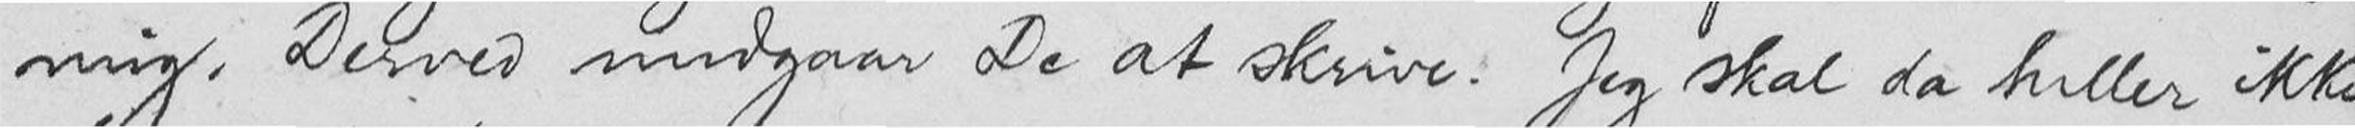mig. Derved undgaar De at skrive. Jeg skal da heller ikke
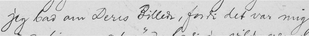jeg bad om Deres Billede, fordi det var mig
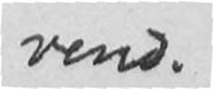vend.
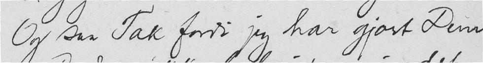Og saa Tak fordi jeg har gjort Dem
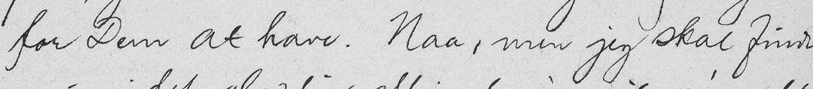for Dem at have. Naa, men jeg skal finde
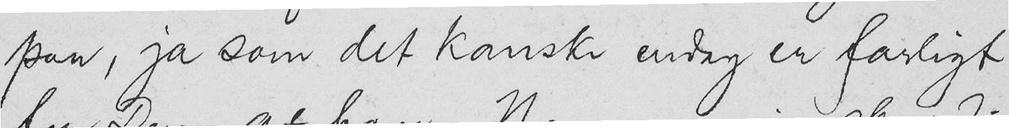paa, ja som det kanske endog er farligt
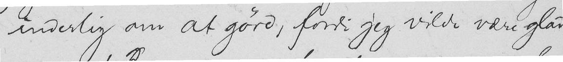inderlig om at gøre, fordi jeg vilde være glad
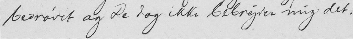bedrøvet og De dog ikke bebrejder mig det.
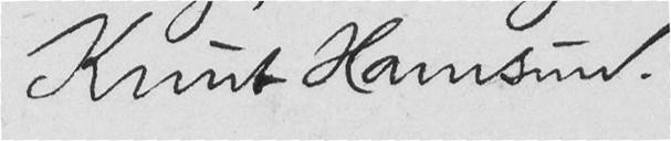Knut Hamsun.
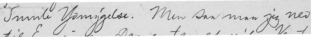Smule Ydmygelse. Men saa maa jeg ned
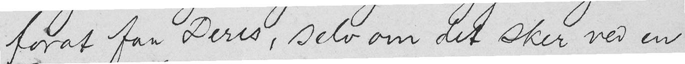forat faa Deres, selv om det sker ved en
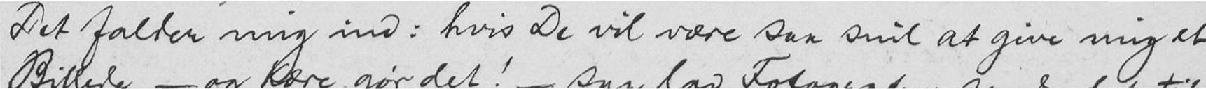Det falder mig ind: hvis De vil være saa snil at give mig et
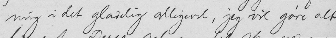mig i det gladelig alligevel, jeg vil gøre alt
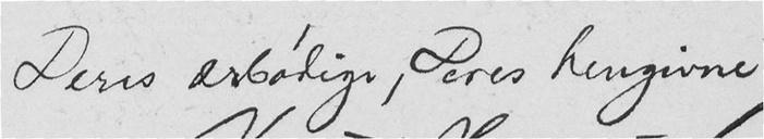Deres ærbødige, Deres hengivne
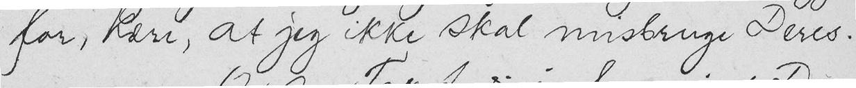for, Kære, at jeg ikke skal misbruge Deres.
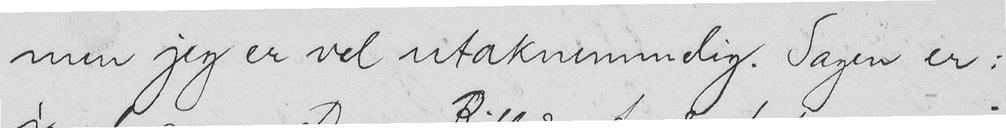men jeg er vel utaknemmelig. Sagen er:
